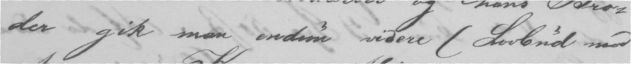der gik man endnu videre (Lovbud mod
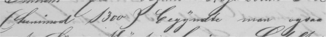(henimod 1300) begyndte man ogsaa
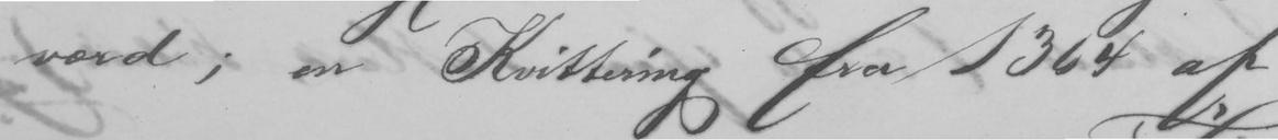værd; en Kvittering fra 1364 af
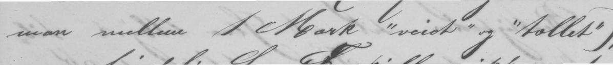man mellem 1 Mark "veiet" og "tællet");
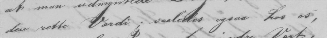den rette Værdi; saaledes ogsaa hos os,
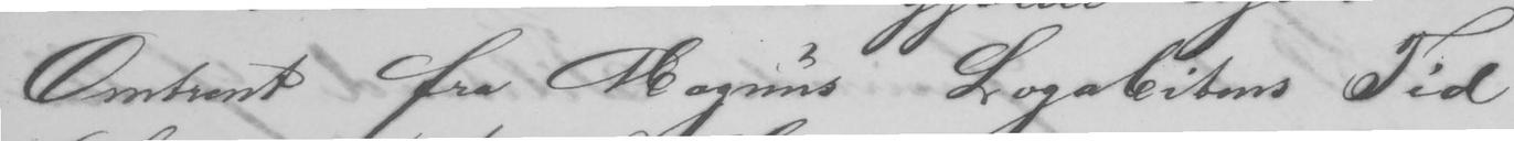Omtrent fra Magnus Logabitons Tid
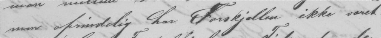men oprindelig har Forskjellen ikke været
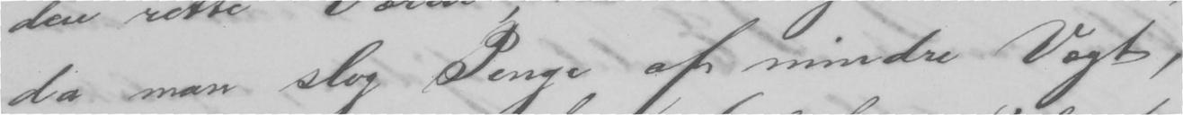da man slog Penge af mindre Vægt,
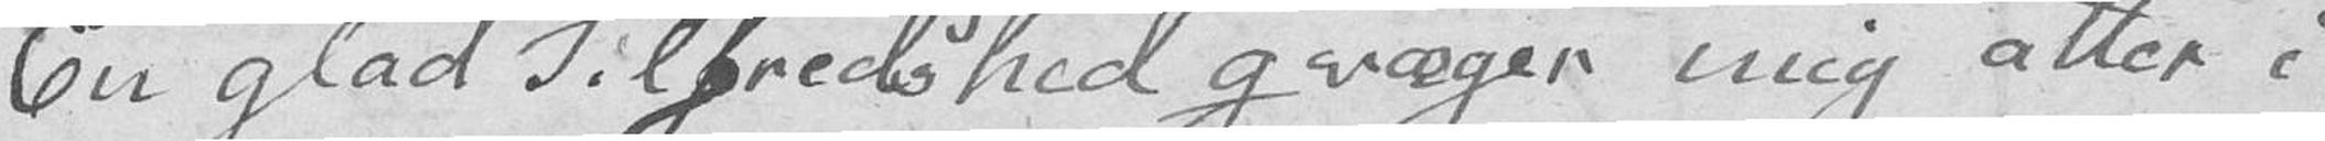En glad Tilfredshed qvæger mig atter i
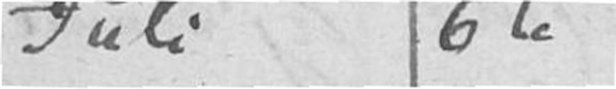Juli 6te
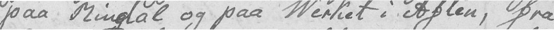paa Rindal og paa Verket i Aften, fra
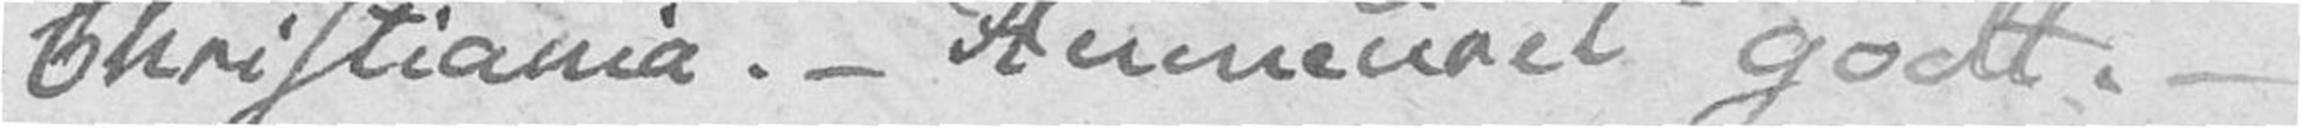Christiania. – Humeuret godt. –
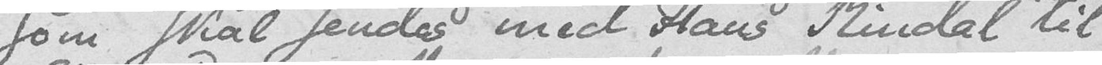som skal sendes med Hans Rindal til
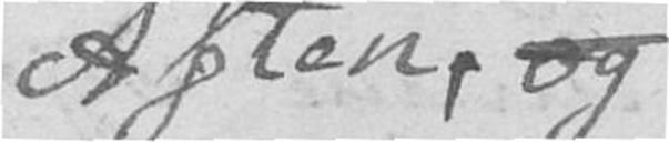Aften, og
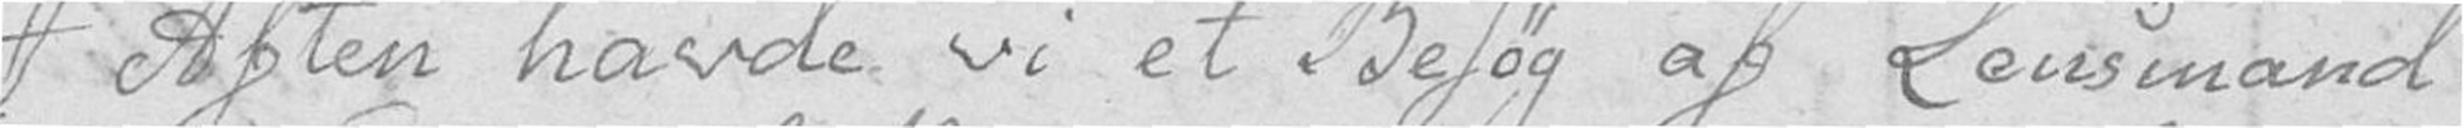I Aften havde vi et Besøg af Lensmand
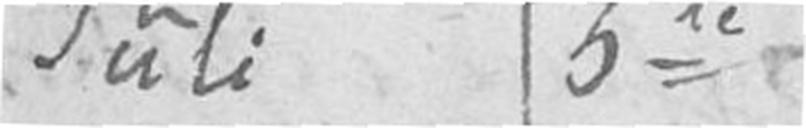Juli 5te
